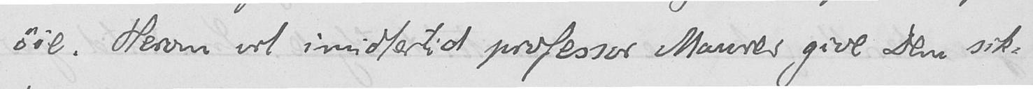øie. Herom vil imidlertid professor Maurer give Dem sik-
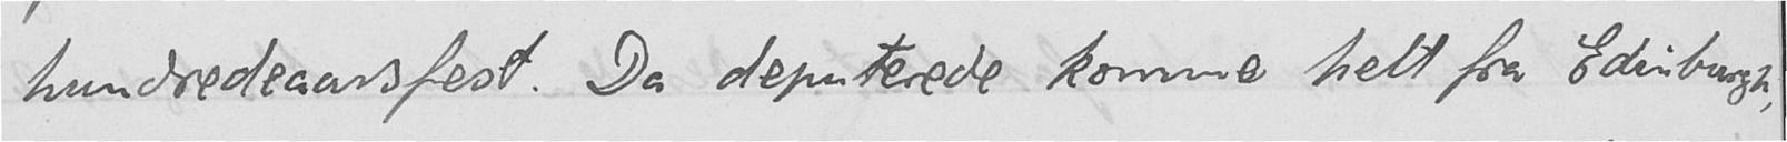hundredeaarsfest. Da deputerede komme helt fra Edinburgh,
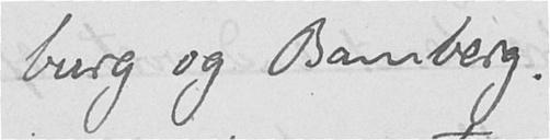burg og Bamberg.
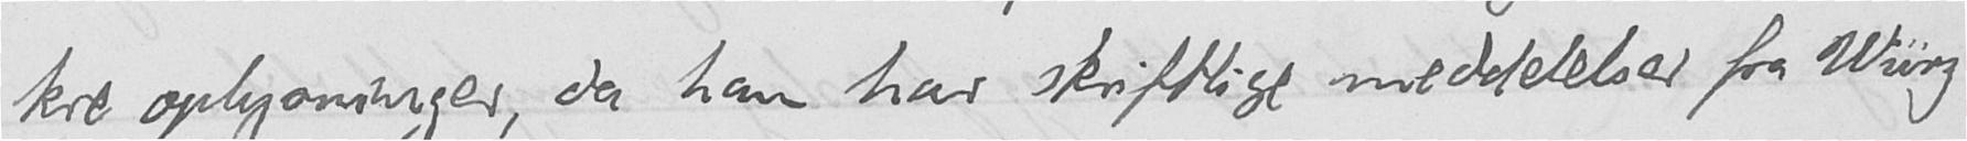kre oplysninger, da han har skriftlige meddelelser fra Würz-
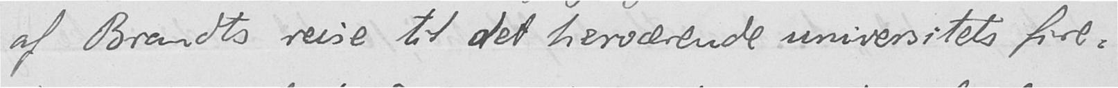af Brandts reise til det herværende universitets fire-
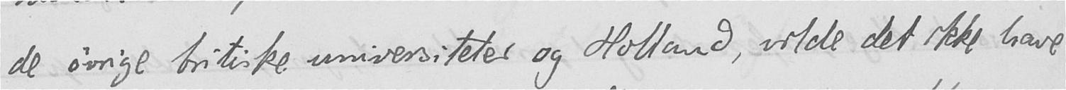de øvrige britiske universiteter og Holland, vilde det ikke have
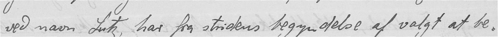ved navn Lutz, har fra stridens begyndelse af valgt at be-
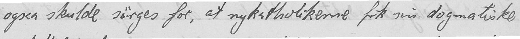ogsaa skulde sørges for, at nykatholikerne fik sin dogmatiske
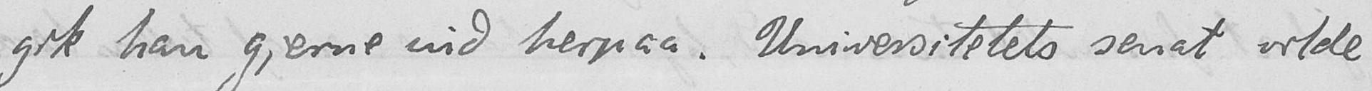gik han gjerne ind herpaa. Universitetets senat vilde
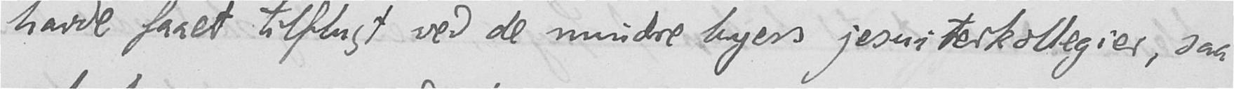havde faaet tilflugt ved de mindre byers jesuitkollegier, saa
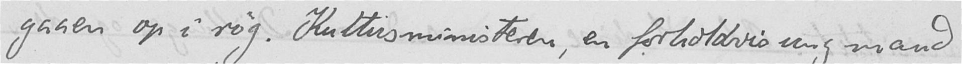gaaen op i røg. Kultusministeren, en forholdvis ung mand
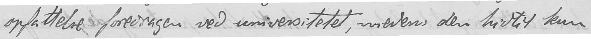opfattelse foredragen ved universitetet, medens den hidtil kun
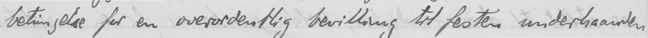betingelse for en overordentlig bevilling til festen underhaanden
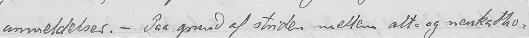anmeldelser. - Paa grund af striden mellem alt- og neukatho-
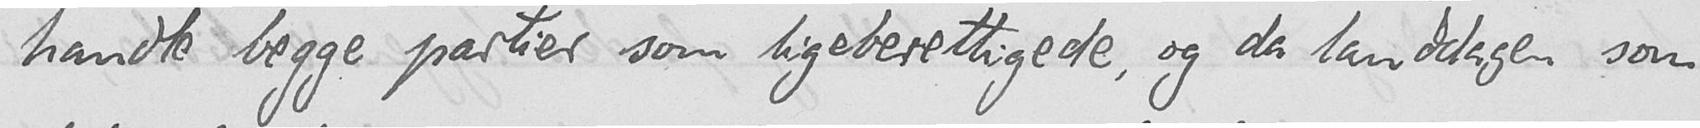handle begge partier som ligeberettigede, og da landdagen som
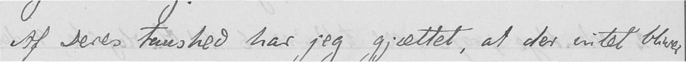Af Deres taushed har jeg gjættet, at der intet bliver
